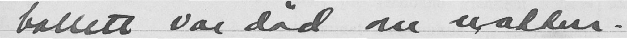Collett var død om natten.
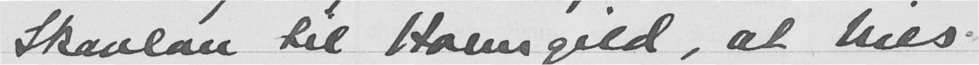Skavlan til Holmgild, at Nils
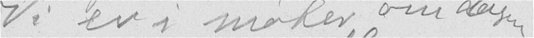Vi er i møter om dagen,
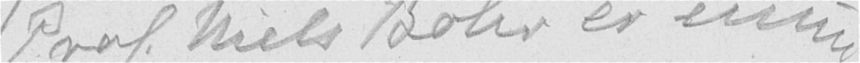Prof. Niels Bohr er ennu
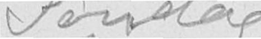Søndag
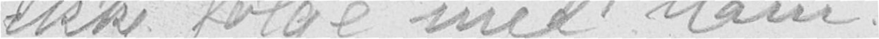ikke følge med ham.
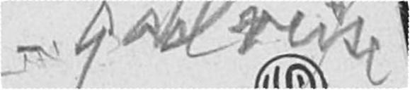- god reise
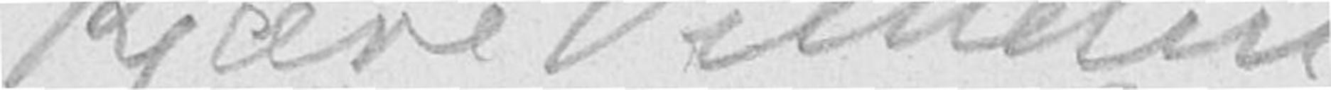Kjære Vilhelm
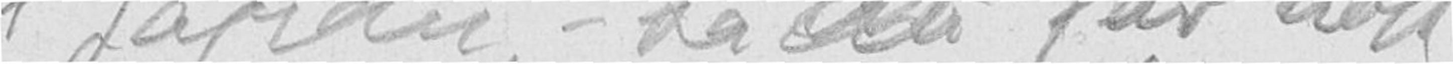i Japan - så du får nok
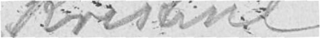Kristine
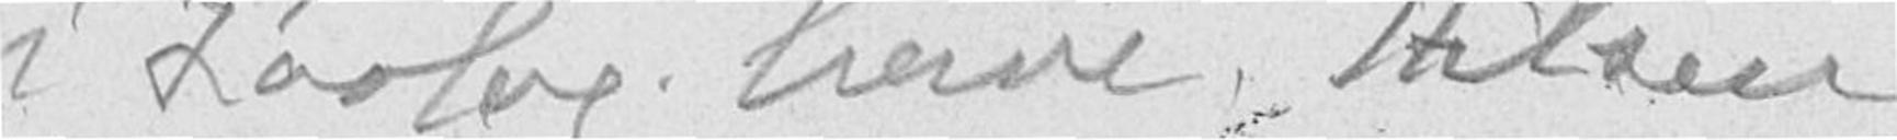i zoolog. have. Hilsen
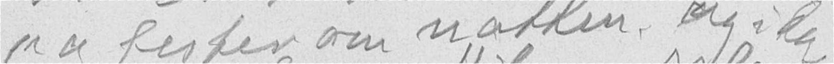på fester om natten, og i dag
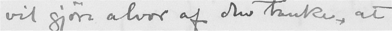vil gjøre alvor af den tanke, at
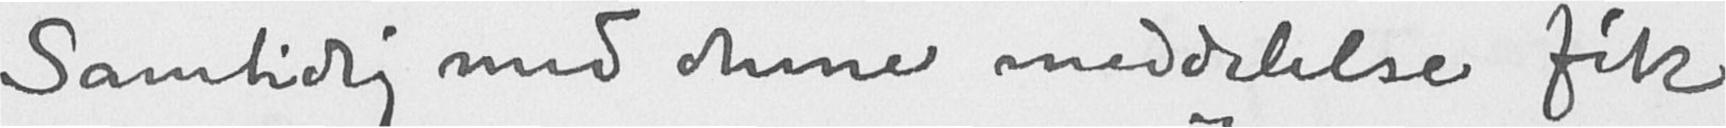Samtidig med denne meddelese fik
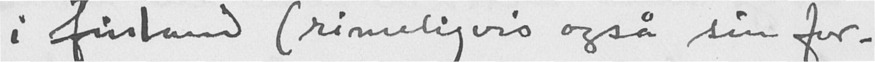i Finland (rimeligvis også sin for-
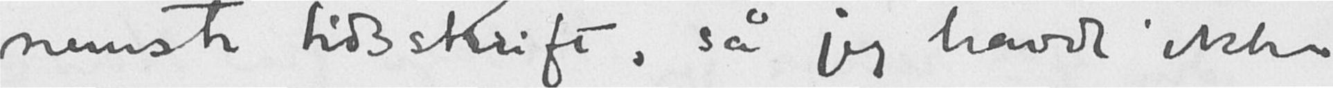nemste tidsstrift, så jeg havde ikke
